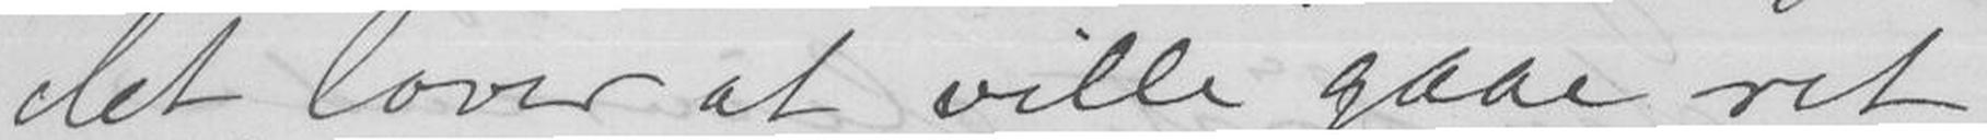det lover at ville gaae ret
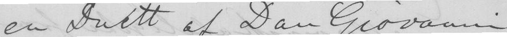en Duett af Don Giovanni
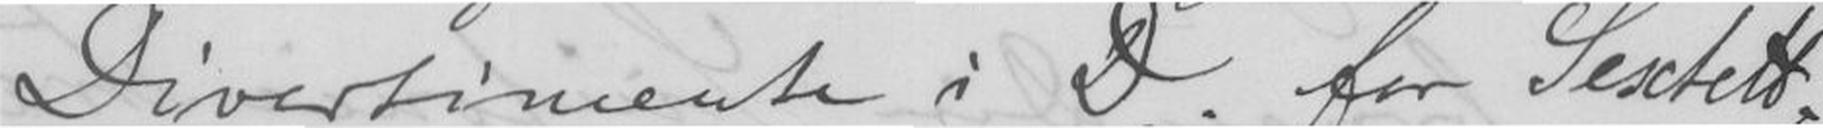Divertimente i D. for Sextett,
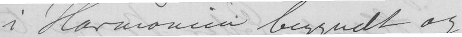i Harmonien begyndt og
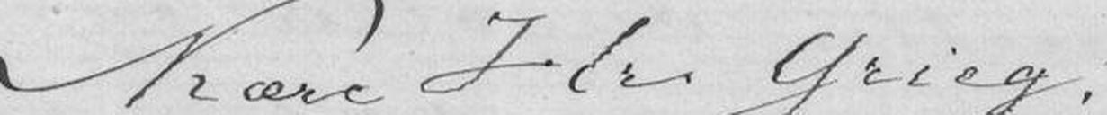Kjære Hr. Grieg !
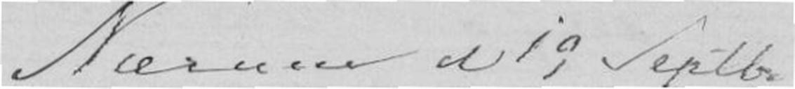Nærum d 19 Sepbr.
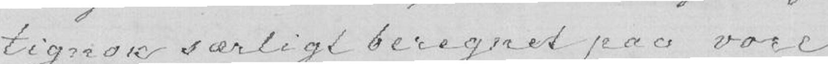tignok særlig beregnet paa vore
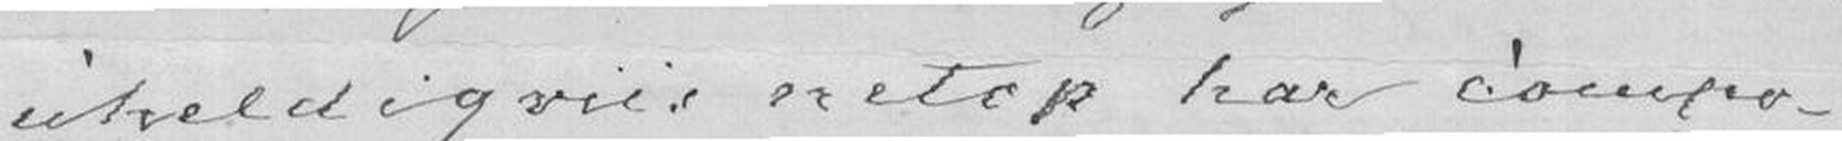uheldigviis netop har compo-
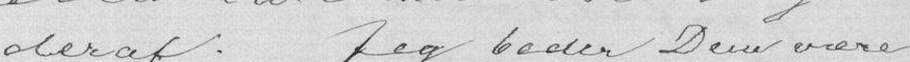deraf. Jeg beder Dem være
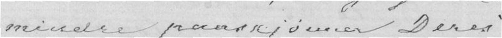mindre paaskjønner Deres
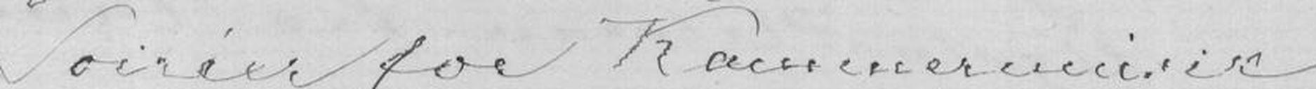Soiréer for Kammermusik
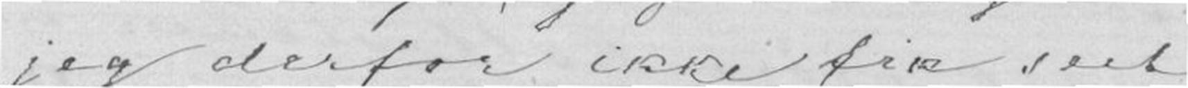jeg derfor ikke fik seet
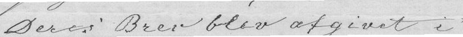Deres Brev blev afgivet i
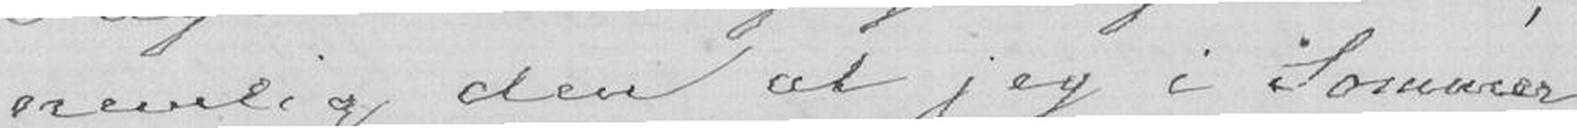nemlig den at jeg i Sommer
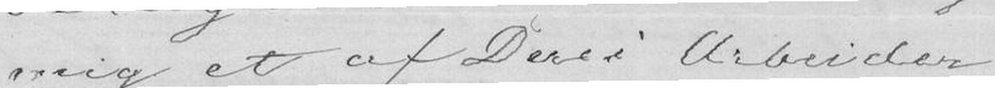mig et af Deres Arbeider,
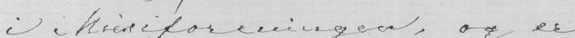i Musicforeningen, og er
